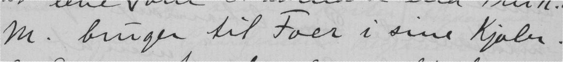M. bruger til Foer i sine Kjoler.
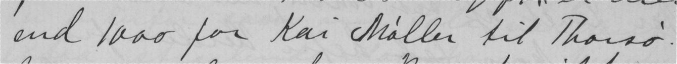end 1000 for Kai Møller til Thorsø.
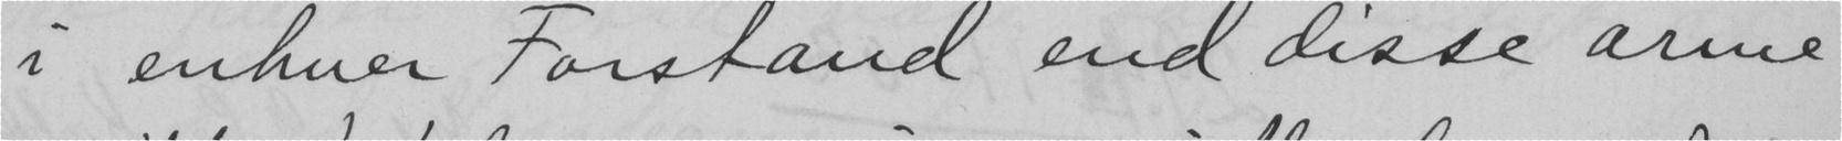i enhver Forstand end disse arme
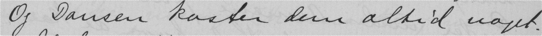Og Dansen koster dem altid noget.
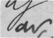av
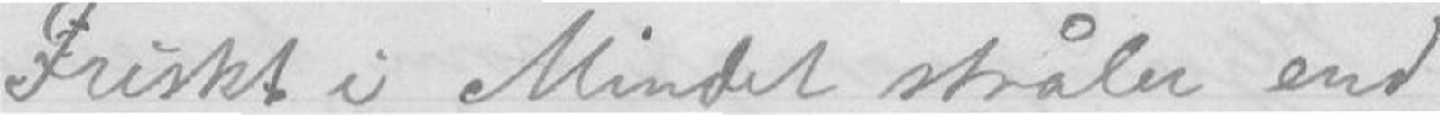Friskt i Mindet stråler end
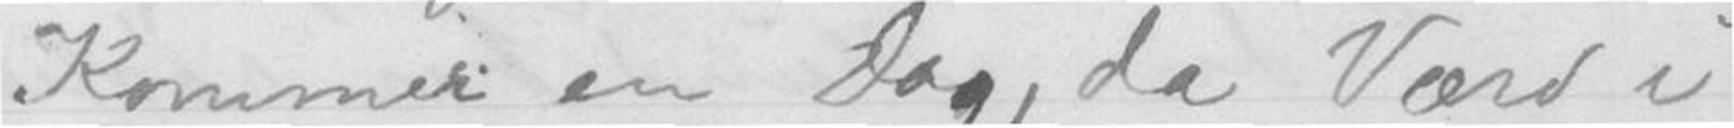Kommer en Dag, Da Værd i
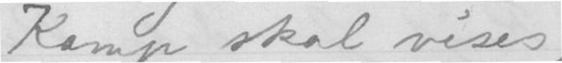Kamp skal vises,
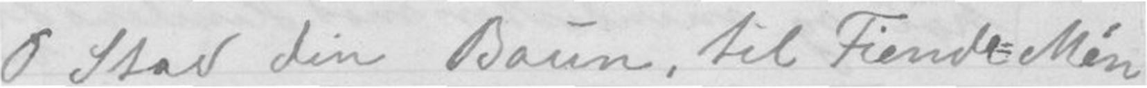O Stav din Baun, til Fiende=Mén
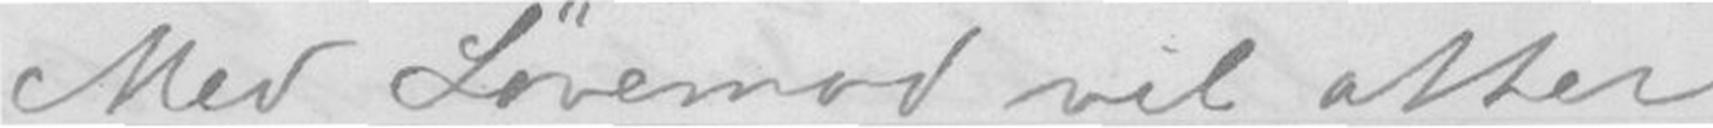Med Løvemod vil atter
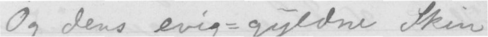Og dens evig=gyldne Skin
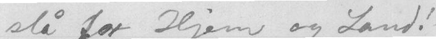slå for Hjem og Land! -
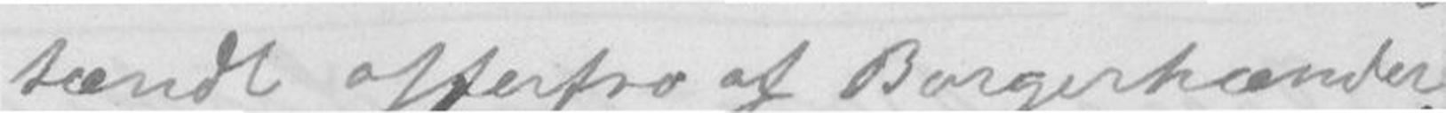tændt offerfro af Borgerhænder.
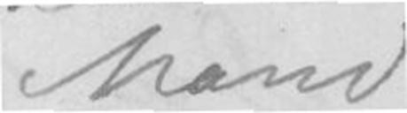Mand
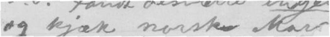og kjæk norske Mar
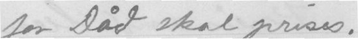for Dåd skal prises.
